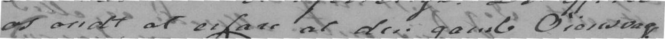os ondt at erfare at den gamle Øiensvag-
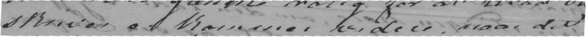skriver ei kommer videre, naar det
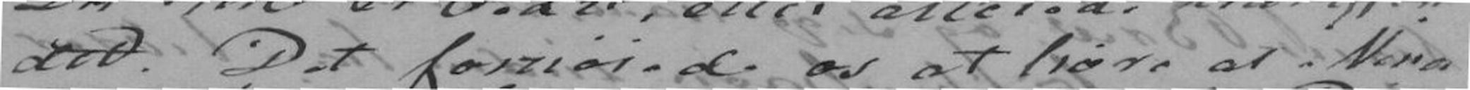det. Det fornøiede os at høre at Nina
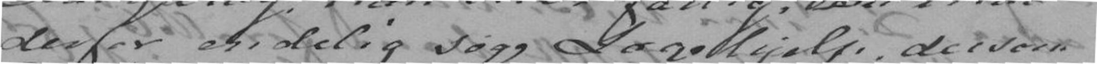derfor endelig søge Lægehjelp, dersom
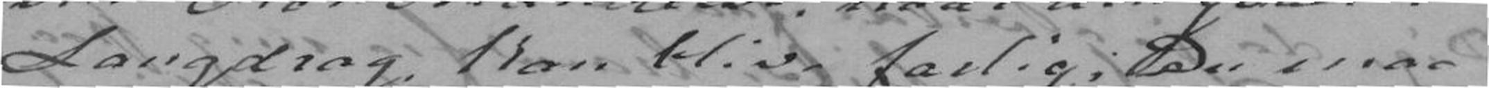Langdrag kan blive farlig; Du maa
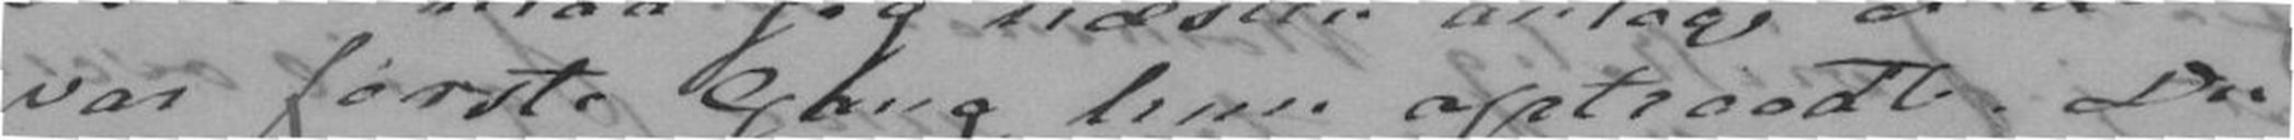var første Gang hun optraadte. Du
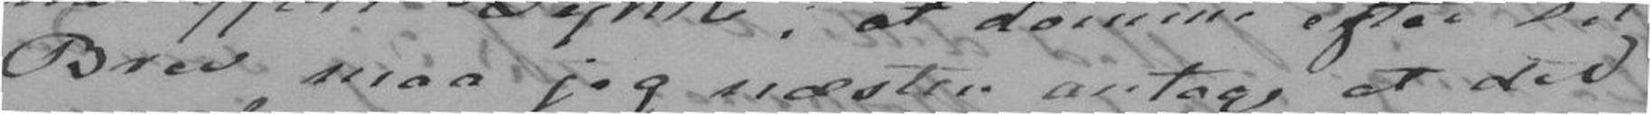Brev maa jeg næsten antage at det
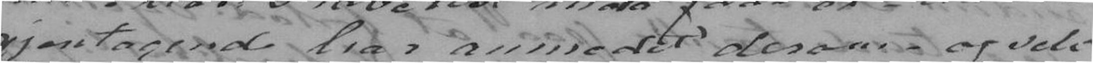gjentagende har anmodet derom, og selv
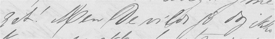get! Men De vilde jo dog ikke
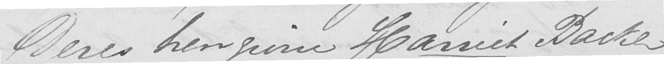Deres hengivne Harriet Backer
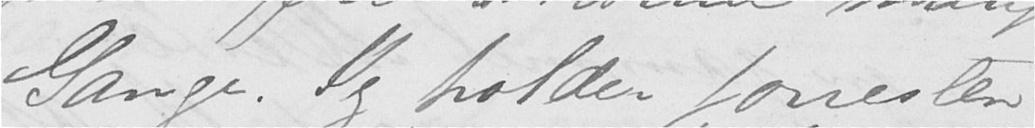Gange. Jeg holder forresten
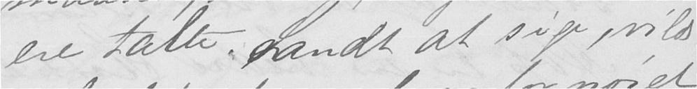ere talte. Sandt at sige, vilde
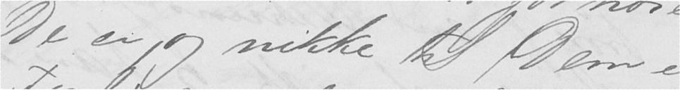De er, og nikke til Dem i
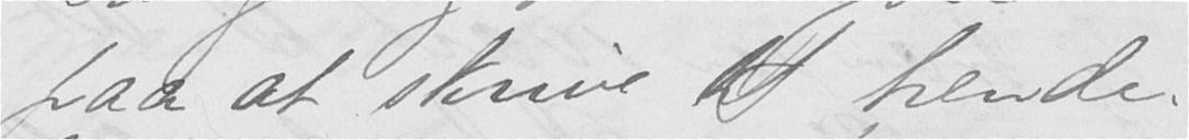paa at skrive til hende.
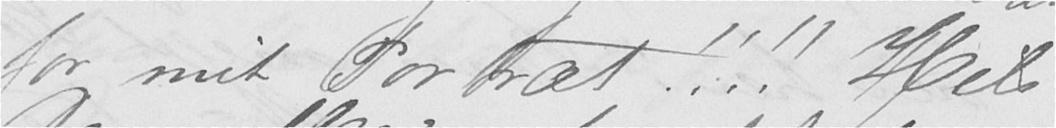for mit Portræt!!!! Hils
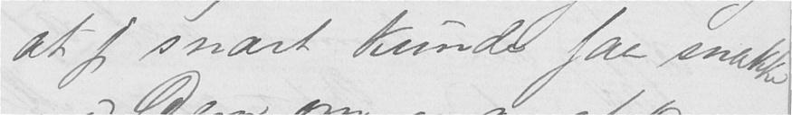at jeg snart kunde faa snakke
